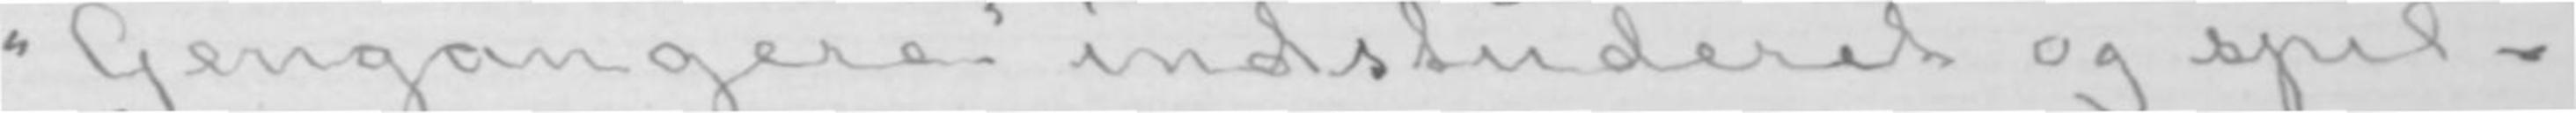«Gengangere» indstuderet og spil-
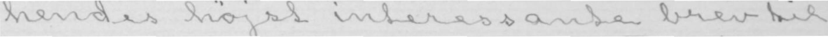hendes højst interessante brev til
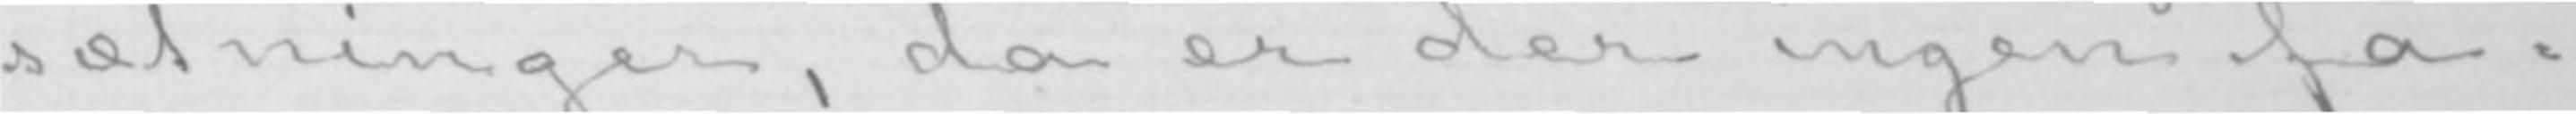sætninger, da er der ingen fa-
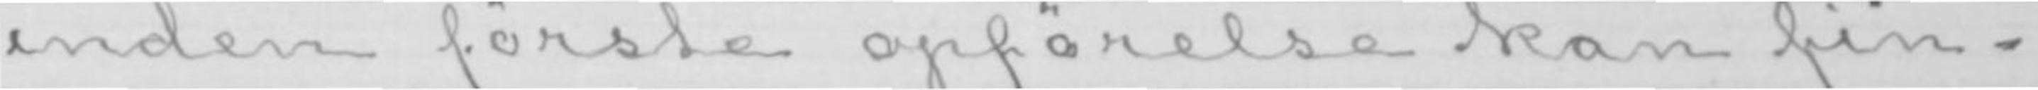inden første opførelse kan fin-
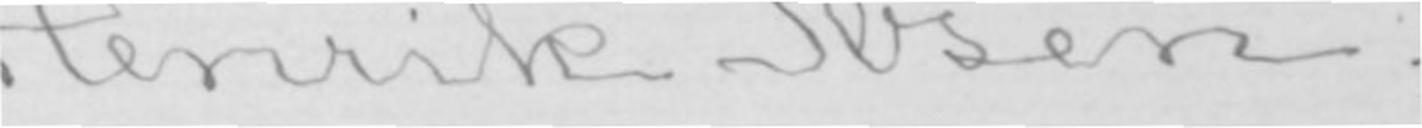Henrik Ibsen.
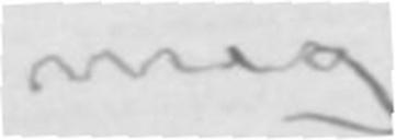mig
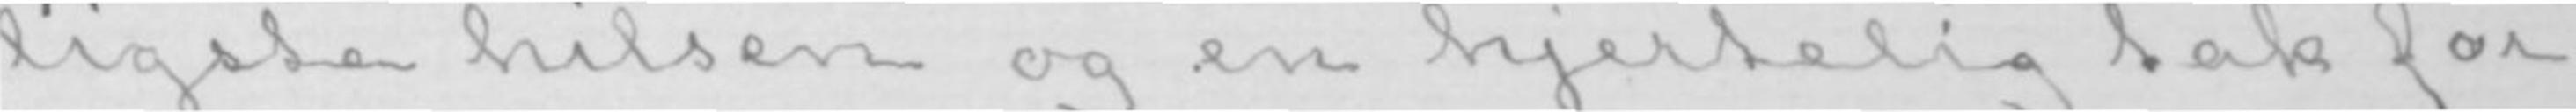ligste hilsen og en hjertelig tak for
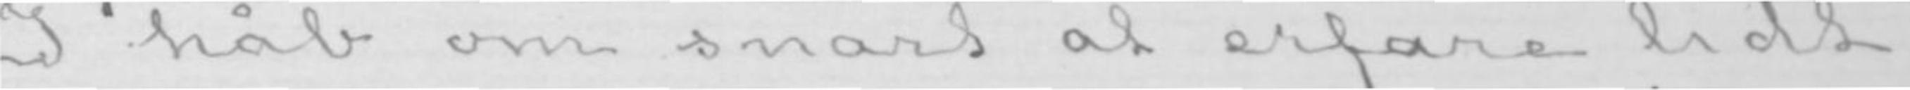I håb om snart at erfare lidt
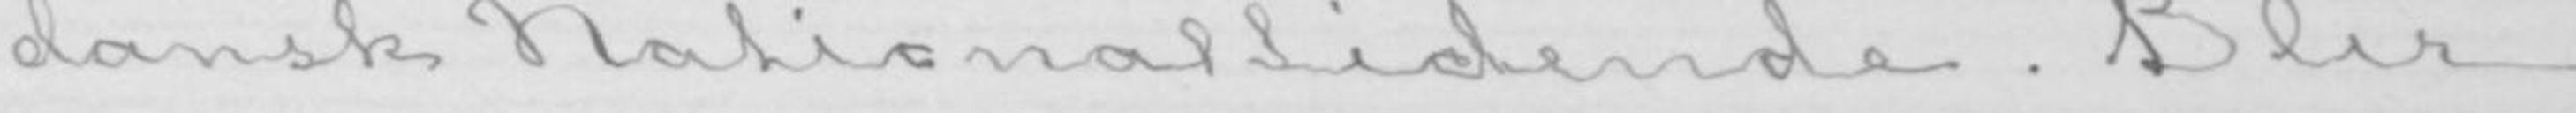dansk Nationaltidende. Blir
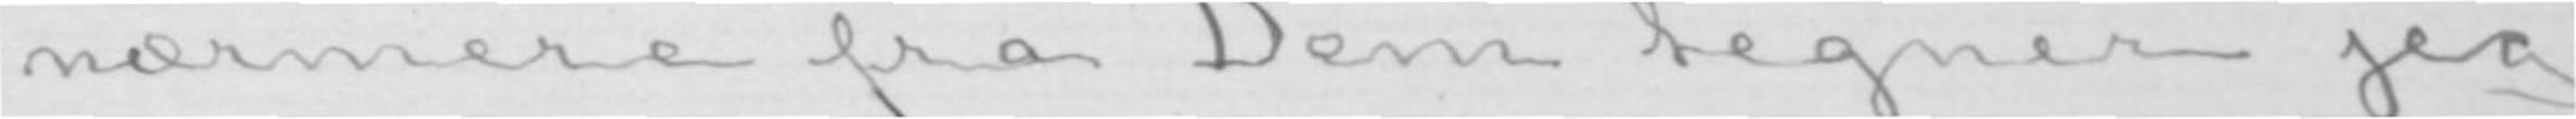nærmere fra Dem tegner jeg
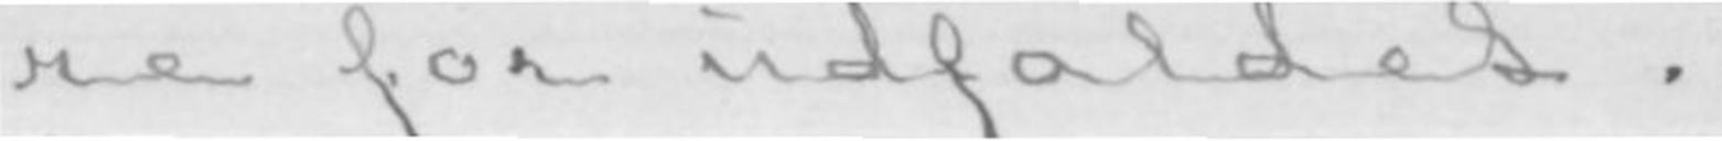re for udfaldet.
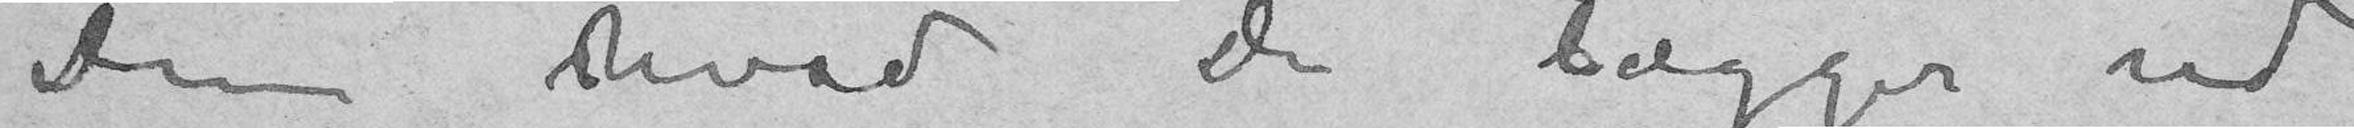Dem hvad De lægger ud
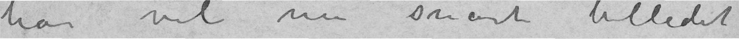har vel nu snart billedet.
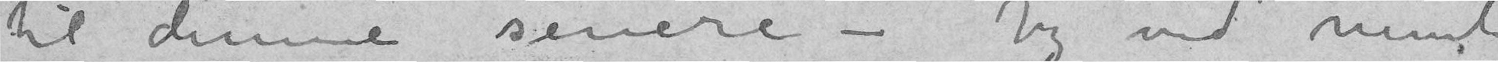til denne senere – Jeg ved nemlig
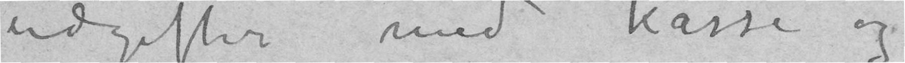udgifter med kasse og
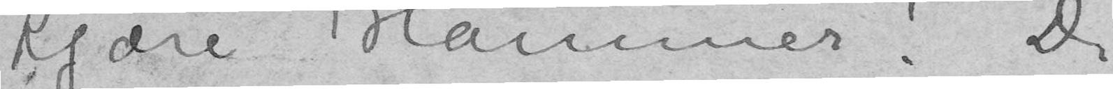Kjære Hammer! De
